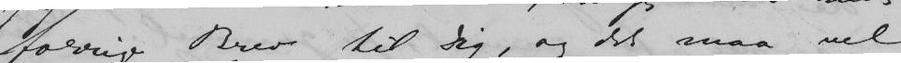forrige Brev til dig, og det maa vel
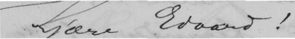Kjære Edvard!
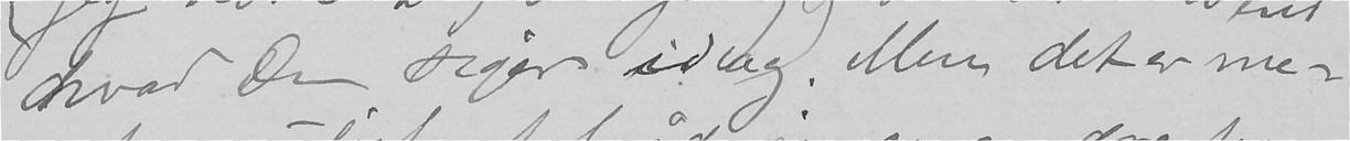hvad Du siger idag. Men det er me-
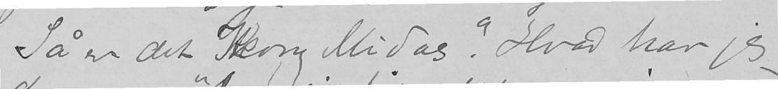Så er det "Kong Midas". Hvad har jeg
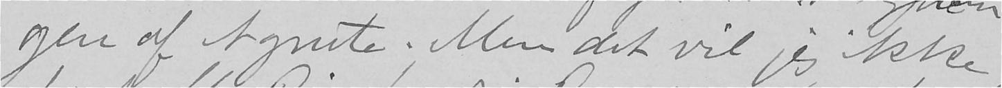gen af Agnete. Men det vil jeg ikke
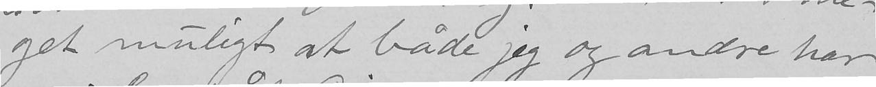get muligt at både jeg og andre har
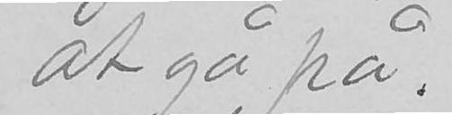at gå på.
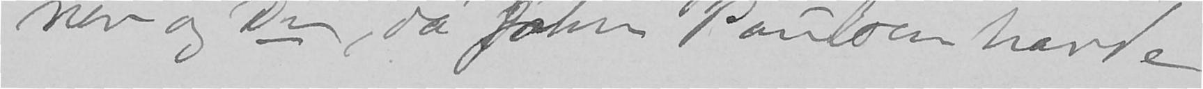ner og Du, da John Paulsen havde
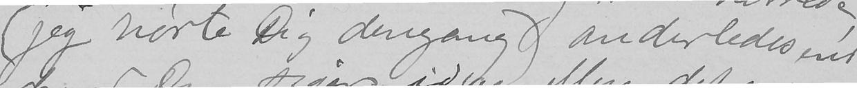(jeg hørte Dig dengang) og anderledes end
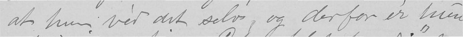at hun vèd det selv, og derfor er hun
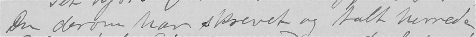Du derom har skrevet og talt hernede,
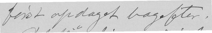først opdaget bagefter.
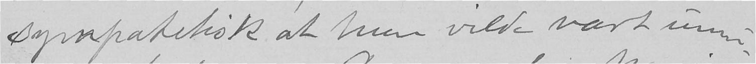sympatetisk at hun vilde vært umu-
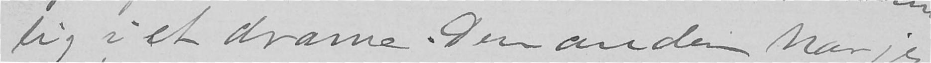lig i et drama. Den anden har jeg
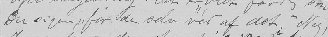Du siger, "før de selv ved af det." Nej,
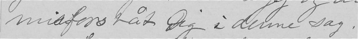misforståt Dig i denne sag.
